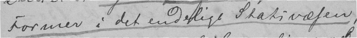Former i det endelige Statsvæsen,
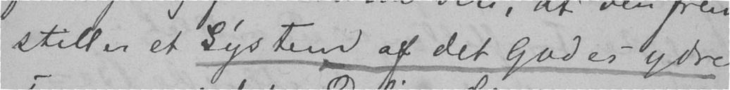stiller et System af det Godes ydre
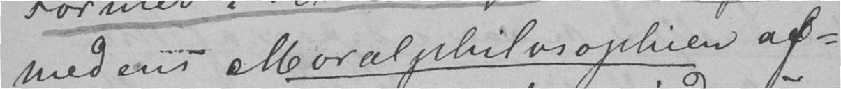medens Moralphilosophien af-
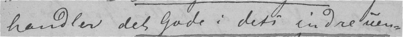handler det gode i dets indre uen-
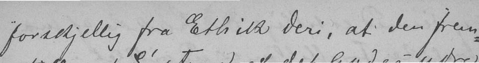forskjellig fra Ethik deri, at den frem-
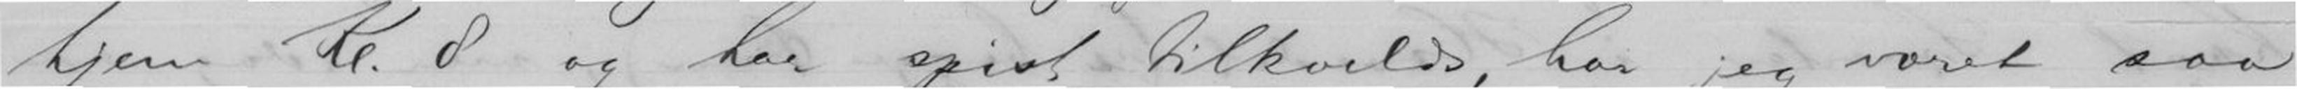hjem Kl.8 og har spist tilkvelds, har jeg været saa
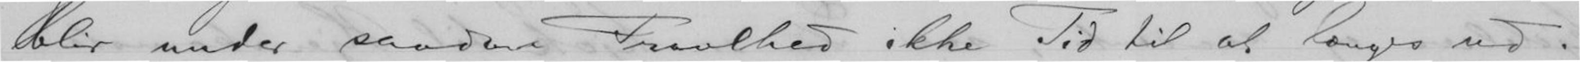blir under saadan Travlhed ikke Tid til at længes ud.
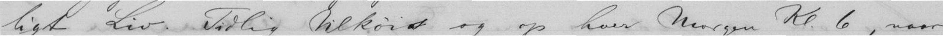ligt Liv. Tidlig tilkøis og op hver Morgen Kl. 6, naar
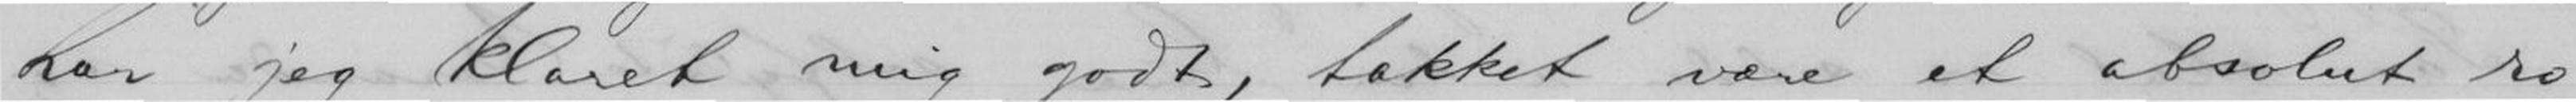har jeg klaret mig godt, takket være et absolut ro-
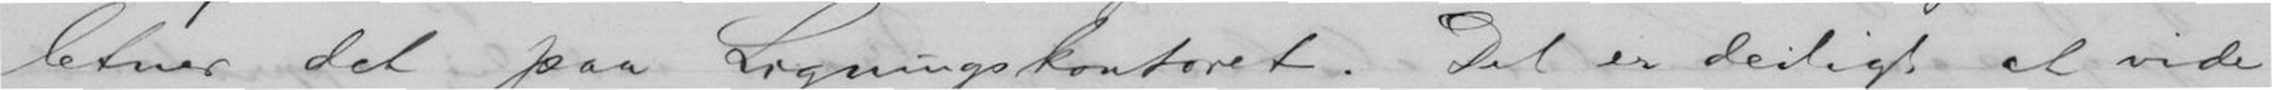letner det paa Ligningskontoret. Det er deiligt at vide
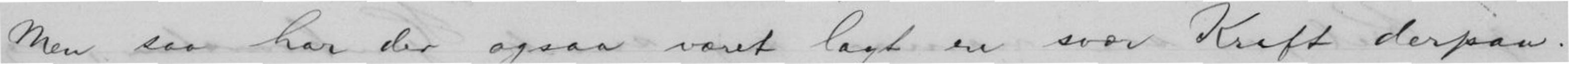Men saa har der ogsaa været lagt en svær Kraft derpaa.
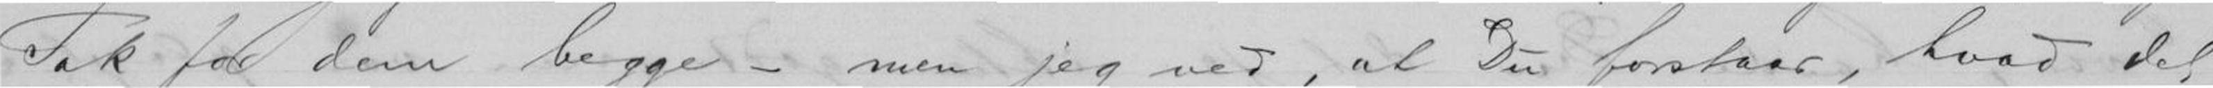Tak for dem begge - men jeg ved, at Du forstaar, hvad det
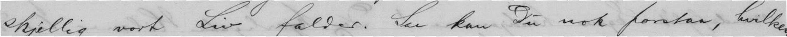skjellig vort Liv falder. Saa kan Du nok forstaa, hvilken
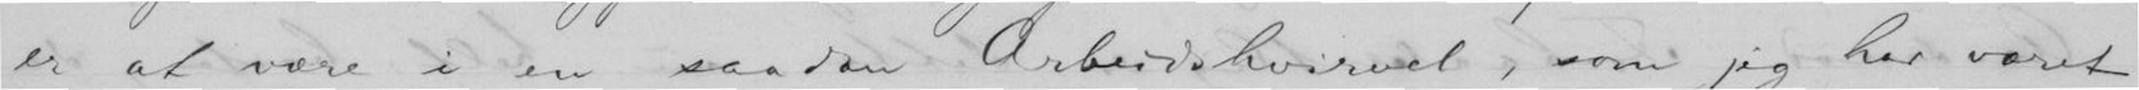er at være i en saadan Arbeidshvirvel, som jeg har været
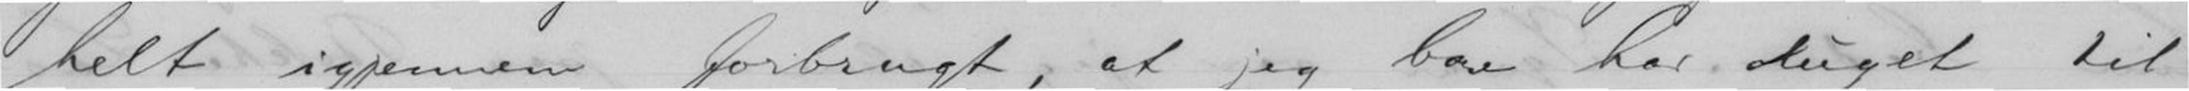helt igjennem forbrugt, at jeg bare har duget til
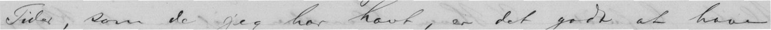Tider, som de jeg har havt, er det godt at have
